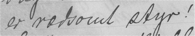er rædsomt styr!
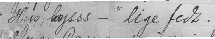"Hys, hysss - " lige fedt.
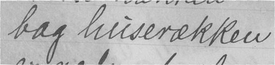bag huserækken
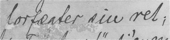forfægter sin ret;
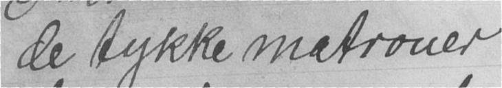de tykke matroner
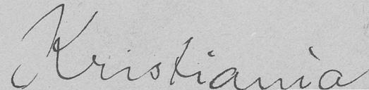Kristiania
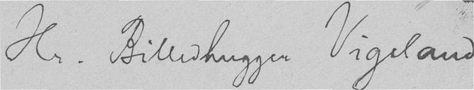Hr. Billedhugger Vigeland
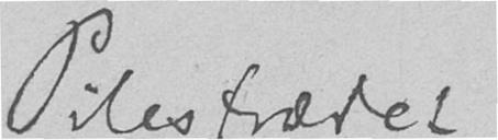Pilestrædet
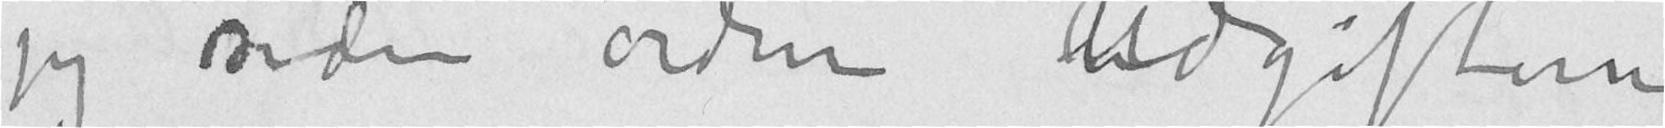jeg siden ordne Udgifterne
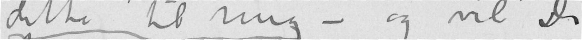dette til mig – og vil De
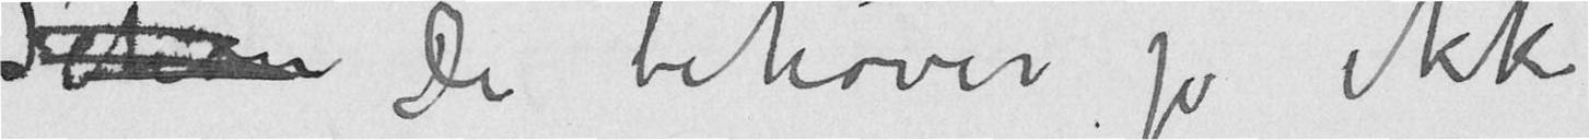Schou De behøver jo ikke
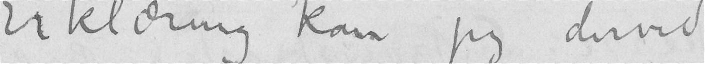Erklæring kan jeg derved
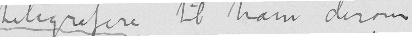telegrafere til ham derom
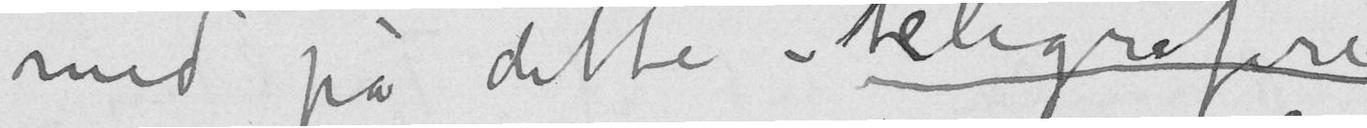med på dette – telegrafere
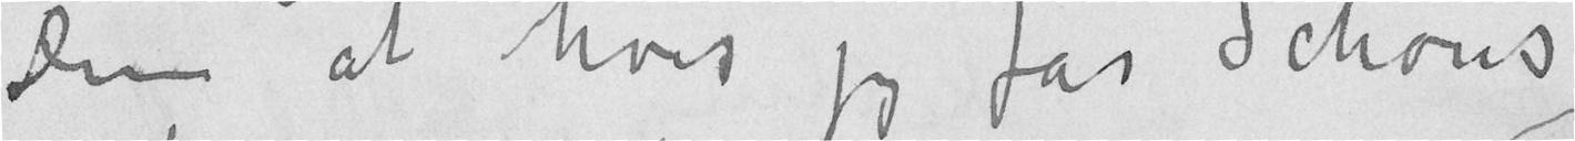Dem at hvis jeg får Schous
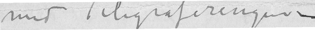med Telegraferingen –
